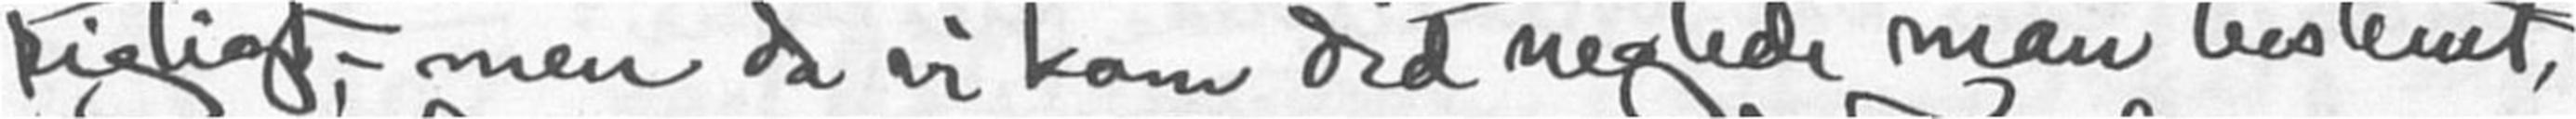rigtigt, - men da vi kom did negtede man bestemt
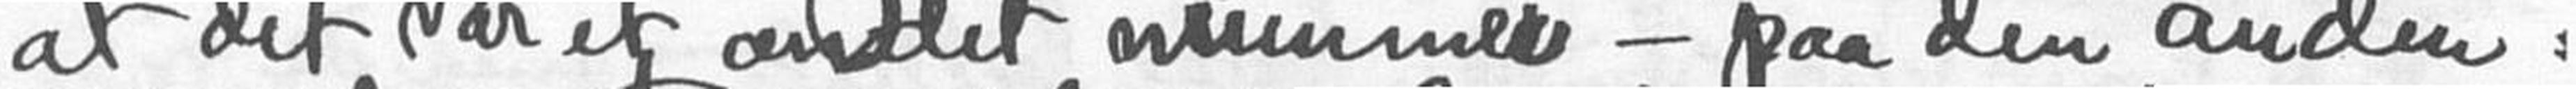at det var et andet nummer - paa den anden
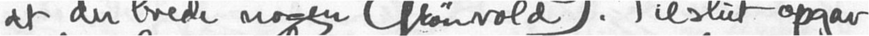at der boede nogen Grønvold).Tilslut opgav
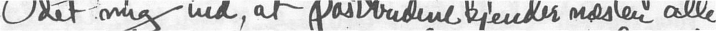det mig ind, at postbudene kjender næsten alle
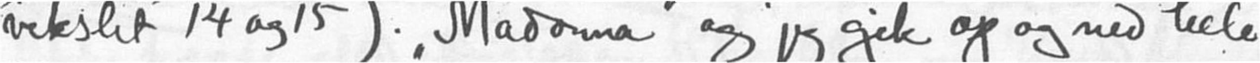vekslet" 14 og 15). "Madonna" og jeg gik op og ned hele
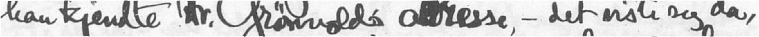han kjendte Hr. Grønvolds adresse, - det viste sig da,
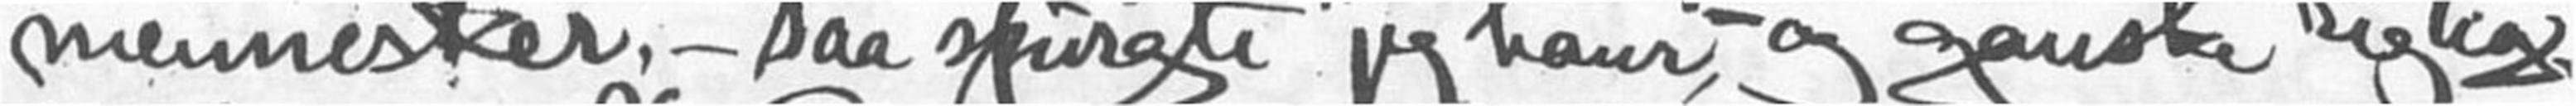mennesker,- saa spurgte jeg ham, - og ganske rigtig -
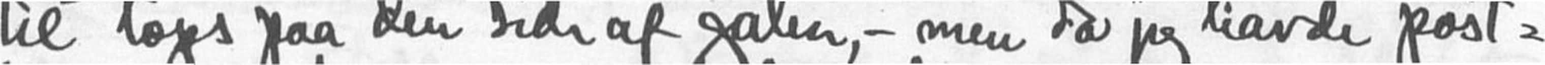til tops paa den side af gaten, - men da jeg havde post-
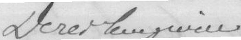Deres hengivne
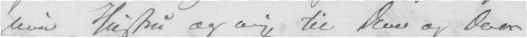min Hustru og mig til Dem og Deres
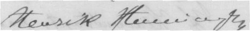Henrik Hennings.
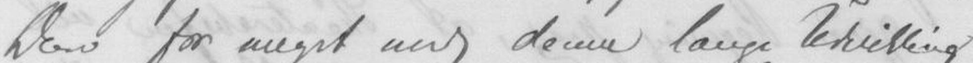Dem for meget med denne lange
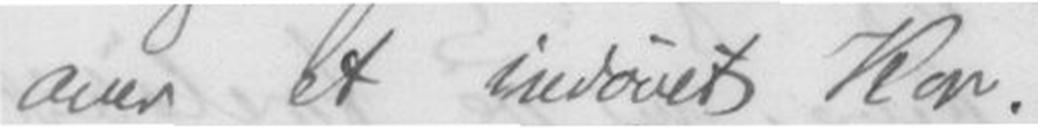over et indøvet Kor.
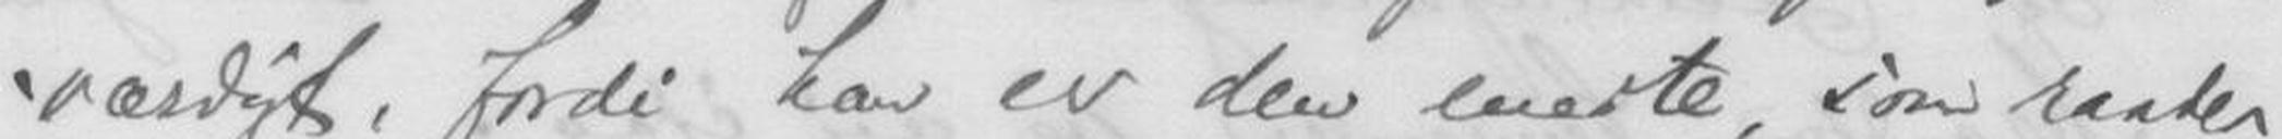værdigt, fordi han er den eneste, som raader
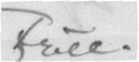Frue.
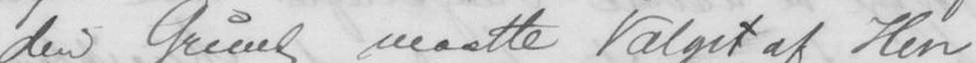den Grund maatte Valget af Herr
